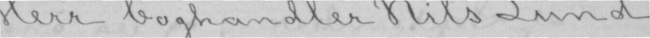Herr boghandler Nils Lund.
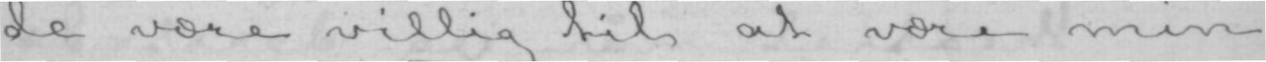de være villig til at være min
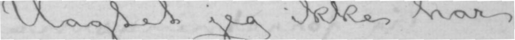Uagtet jeg ikke har
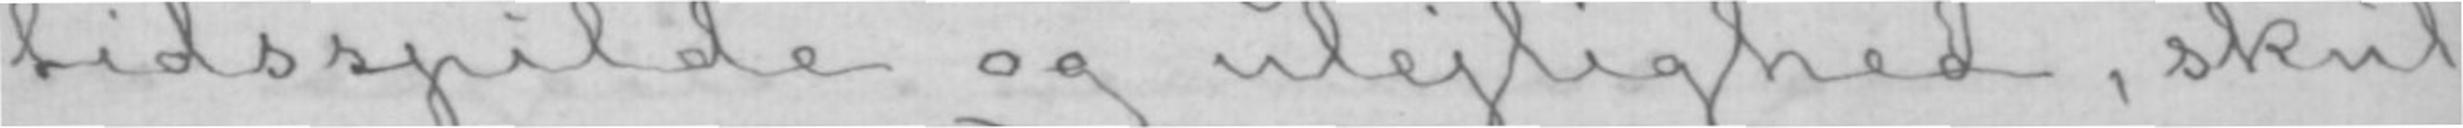tidsspilde og ulejlighed, skul-
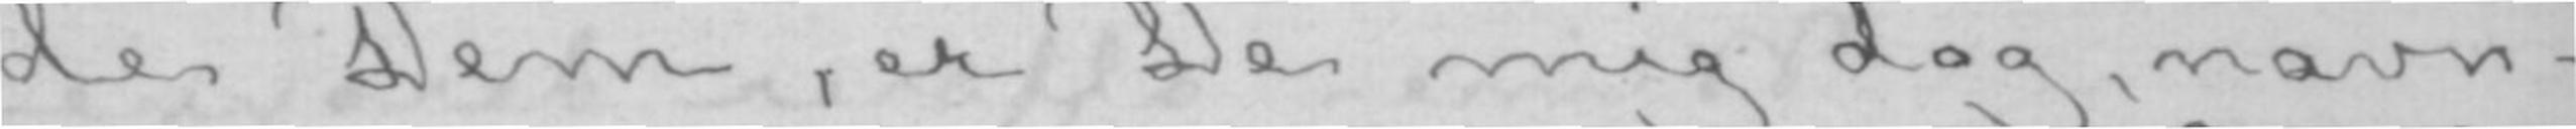de Dem, er De mig dog, navn-
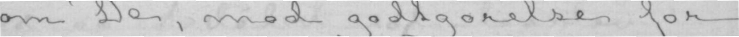om De, mod godtgørelse for
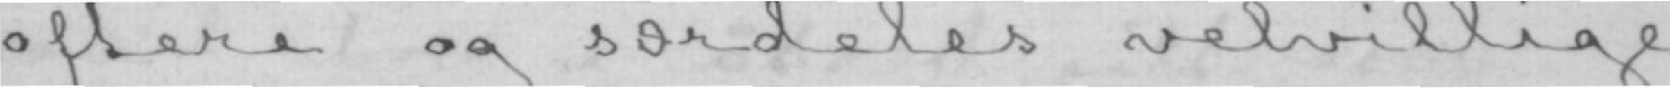oftere og særdeles velvillige
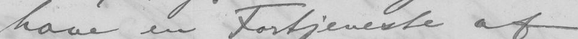have en Fortjeneste af
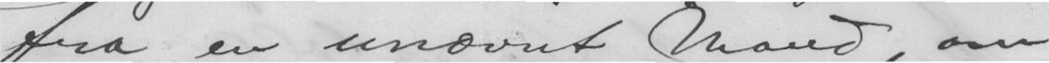fra en unævnt Mand, om
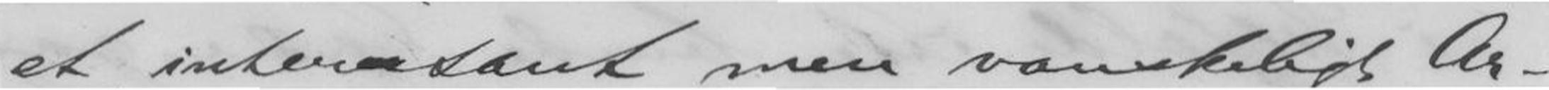et interessant men vanskeligt Ar-
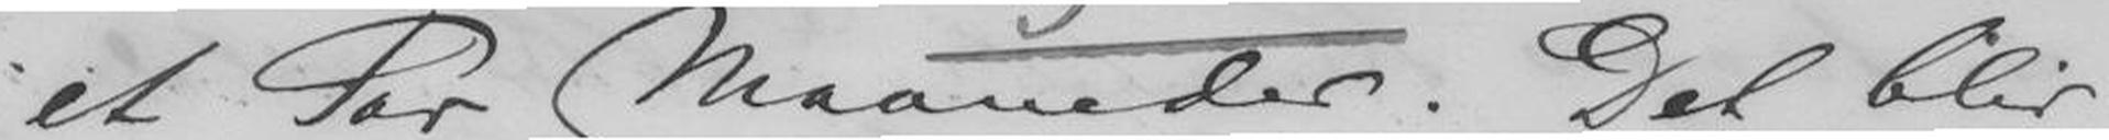et Par Maaneder. Det blir
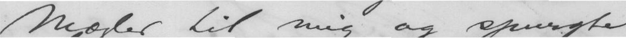Mægler til mig og spurgte
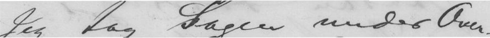Jeg tog Sagen under Over-
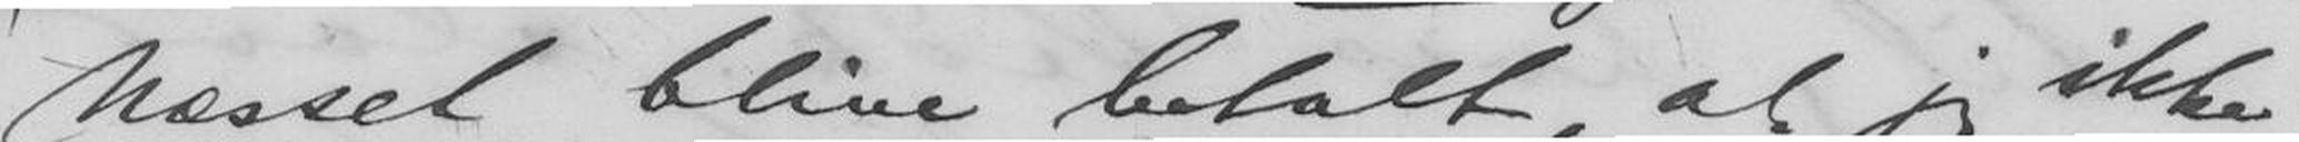Næssset blive betalt, at jeg ikke
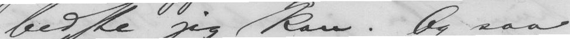bedste jeg kan. Og saa
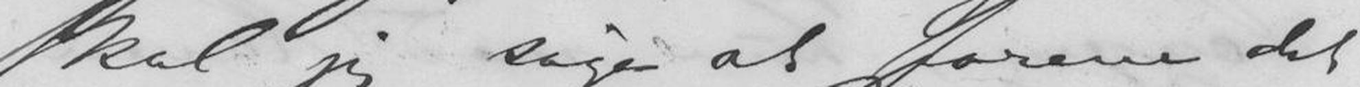skal jeg søge at forene det
